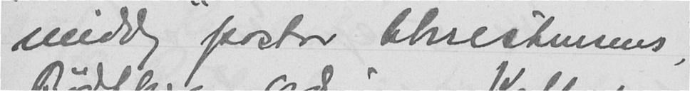middag pastor Christensens,
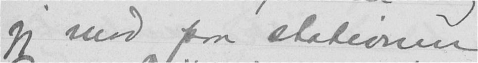jeg mod paa stationen
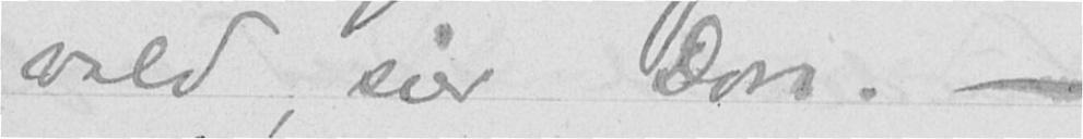vold, sier Don. -
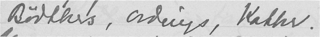Bødtkers, Ordings, Kathr.
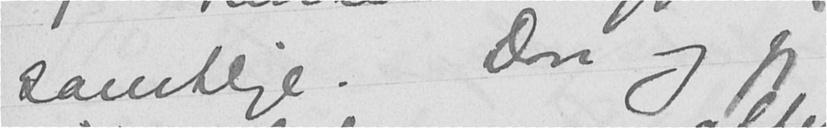samtlige. Don og jeg
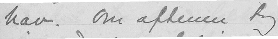hav. Om aftenen tog
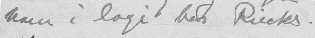ham i logi hos Riecks.
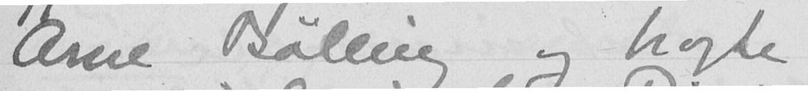Arne Bølling og bragte
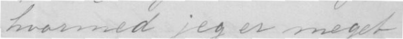hvormed jeg er meget
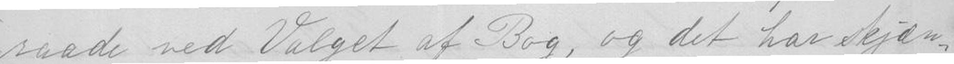raade ved Valget af Bog, og det har skjæn-
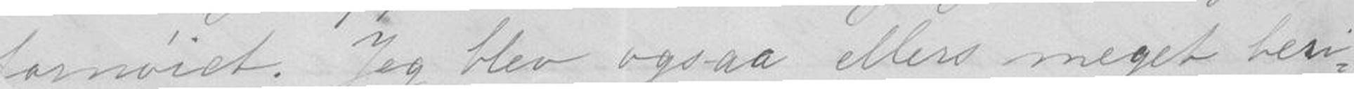fornøiet. Jeg blev ogsaa ellers meget hen-
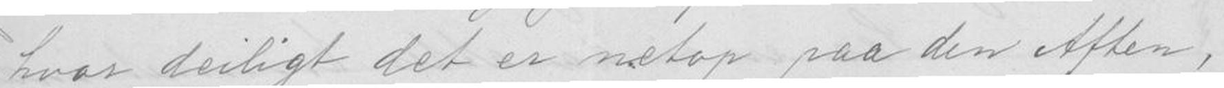hvor deiligt det er netop paa den Aten,
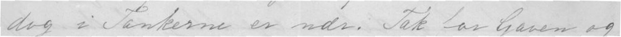dog i Tankerne er nær. Tak for Gaven og
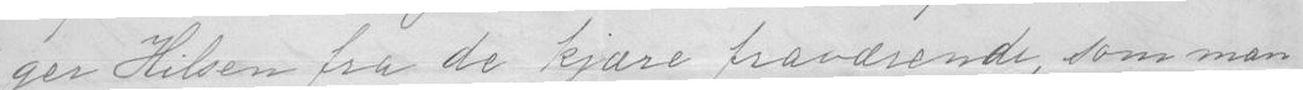ger Hilsen fra de kjære fraværende , som man
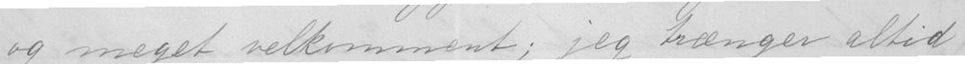og meget velkomment; jeg trænger altid
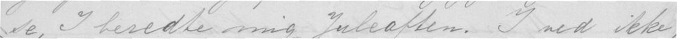se, I beredte mig Juleaften. I ved ikke,
